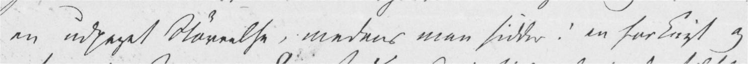en udpreget Størrelse, medens man sidder i en forknyt og
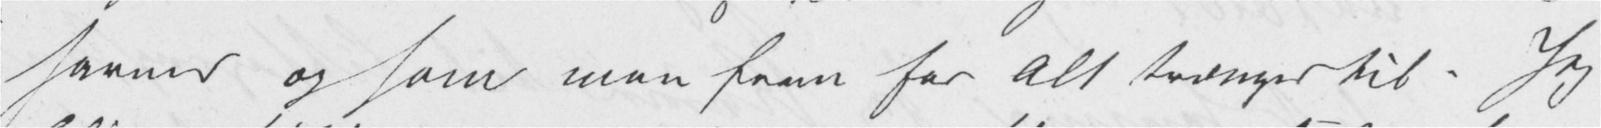savner og som man frem for Alt trænger til. Jeg
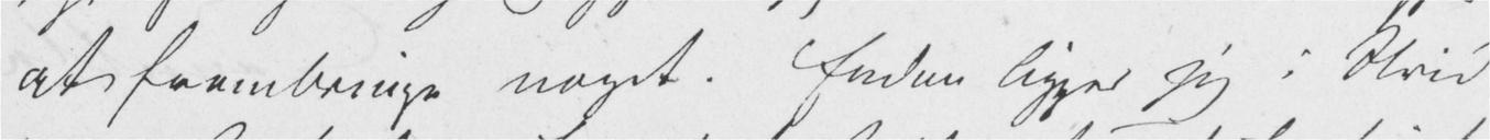at frembringe noget. Endnu ligger jeg i Strid
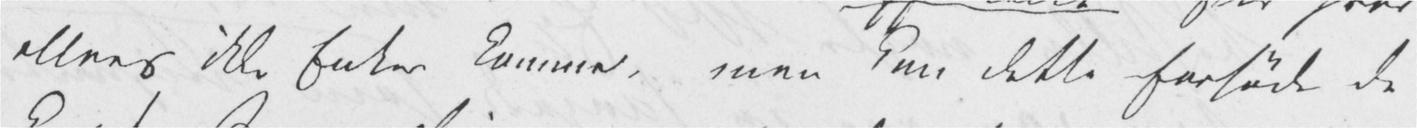ellers ikke Enker komme, men kan dette forsøde de
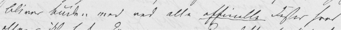bliver buden med ved alle officielle Fester hvor
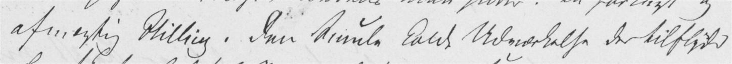afmægtig Stilling. Den Smule kolde Udmærkelse der tilflyder
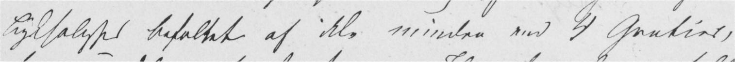Lyksalighed befolket af ikke mindre end 4 Gratier,
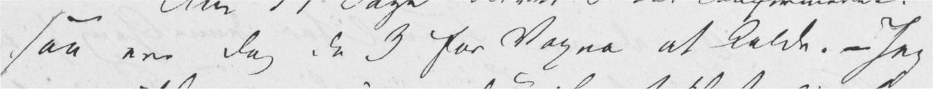saa ere dog de 3 for Voksne at kalde. – Jeg
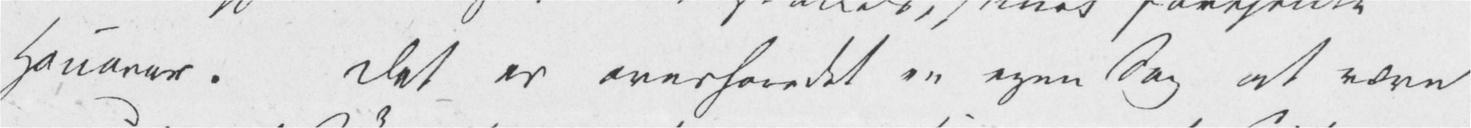Honorar. Det er overhovedet en egen Sag at være
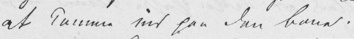at komme ind paa den Bane.
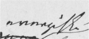energiske
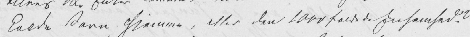kolde Savn hjemme, eller den 1000foldede Ensomhed?
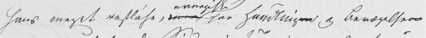Hans meget rastløse, snare paa Handlingen og Bevægelsen
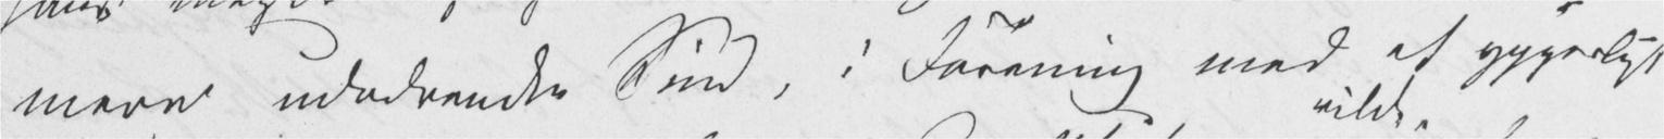mere udadvendte Sind, i Forening med et ypperligt
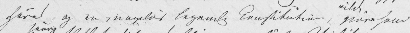Hoved, og en mageløs legemlig Konstitution, gjøre ham
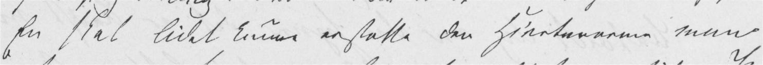En skal lidet kunne erstatte den Hjertevarme man
